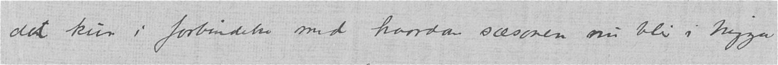det kun i forbindelse med hvordan sæsonen nu blir i Nizza
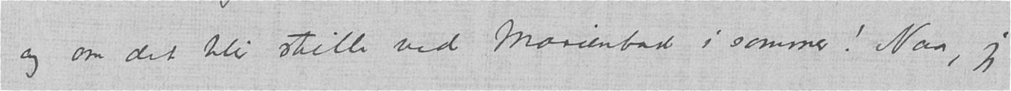og om det blir stille ved Marienbad i sommer! Naa, jeg
